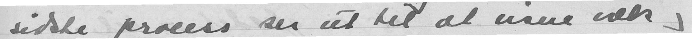sidste process ser ut til at visne væk og
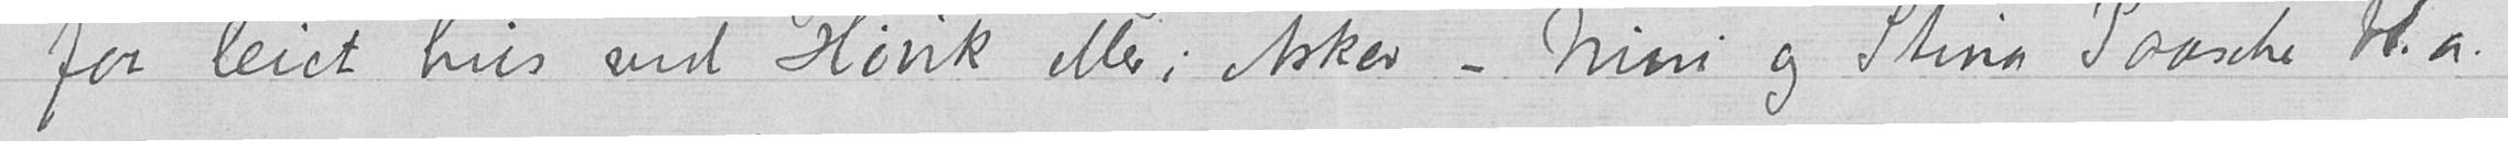faa leiet hus ved Høvik eller i Asker – Nini og Stina Paasche bl.a.
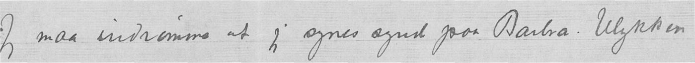Jeg maa indrømme at jeg synes synd paa Barbra. Ulykken
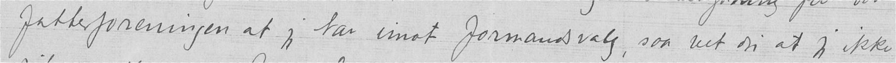fatterforeningen at jeg tar imot formandsvalg, saa vet du at jeg ikke
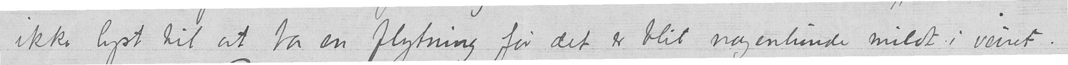ikke lyst til at ta en flytning før det er blit nogenlunde mildt i veiret.
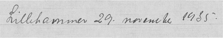Lillehammer 29. november 1935.
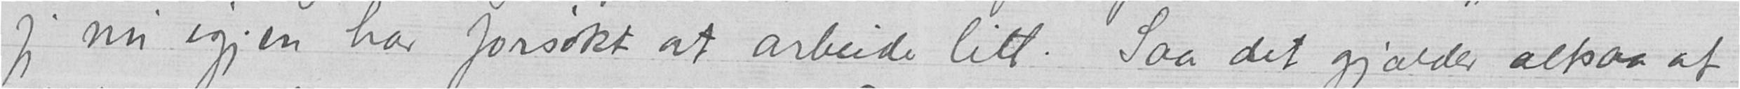jeg nu igjen har forsøkt at arbeide litt. Saa det gjælder altsaa at
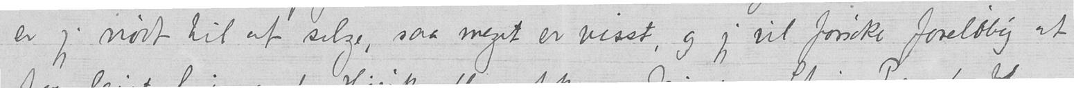er jeg nødt til at selge, saa meget er visst, og jeg vil forsøke foreløbig at
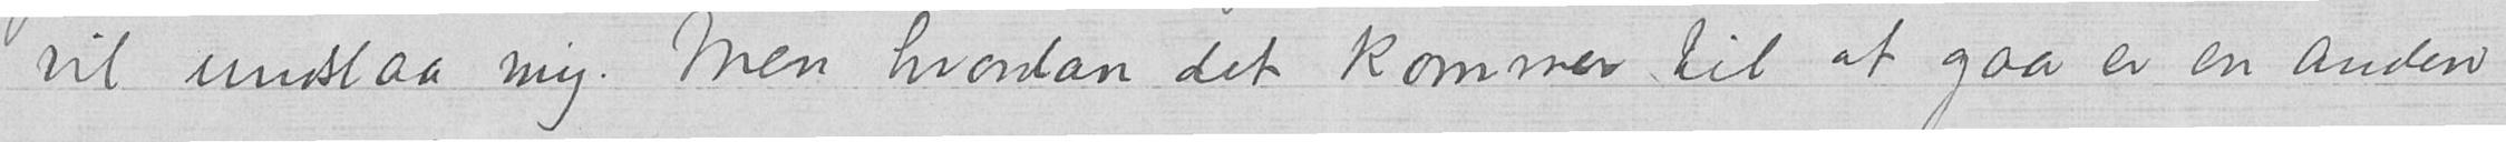vil undslaa mig. Men hvordan det kommer til at gaa er en anden
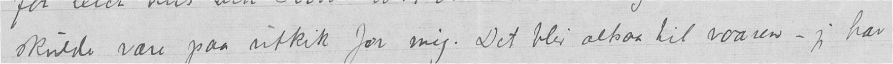skulde være paa utkik for mig. Det blir altsaa til vaaren — jeg har
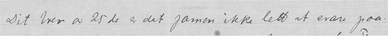Dit brev av 25de er det jamen ikke lett at svare paa.
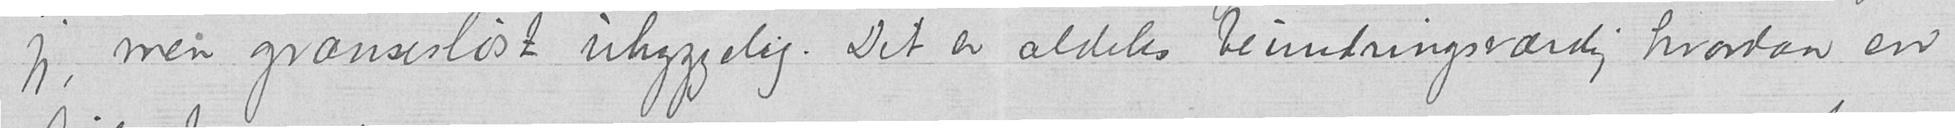jeg, men grænsesløst uhyggelig. Det er aldeles beundringsværdig hvordan en
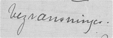begrænsninger:
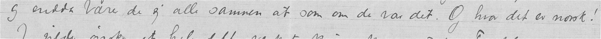og endda bærer de sig alle sammen at som om de var det. Og hvor det er norsk!
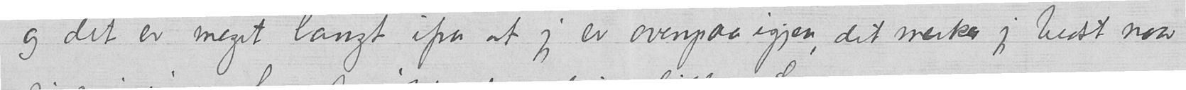og det er meget langt ifra at jeg er ovenpaa igjen, det merker jeg bedst naar
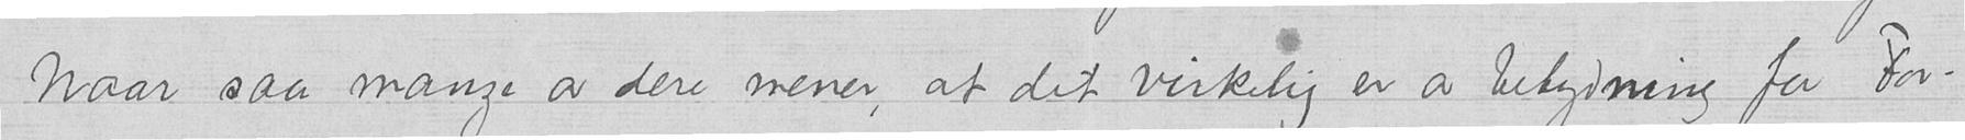Naar saa mange av dere mener, at det virkelig er av betydning for For-
